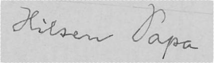Hilsen Papa
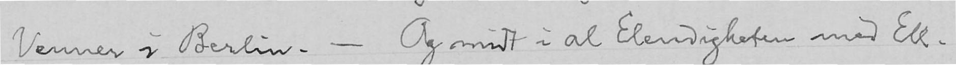Venner i Berlin. - Og midt i al Elendigheten med Ell.
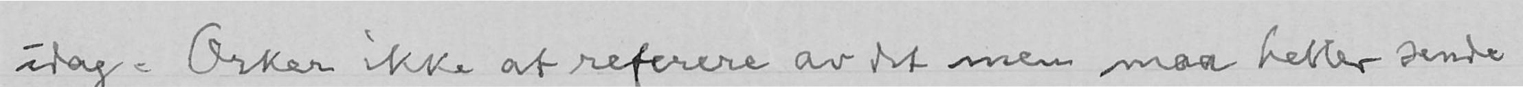idag. Orker ikke at referere av det men maa heller sende
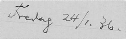Fredag 24/1. 36.
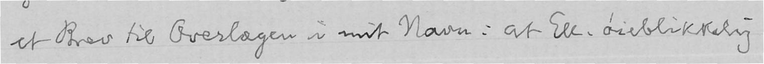et Brev til Overlægen i mit Navn: at Ell. øieblikkelig
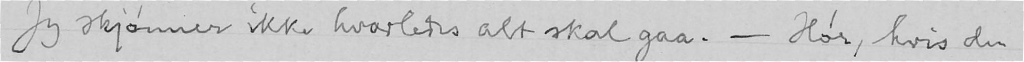Jeg skjønner ikke hvorledes alt skal gaa. - Hør, hvis du
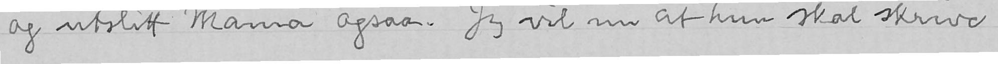og utslitt Mama ogsaa. Jeg vil nu at hun skal skrive
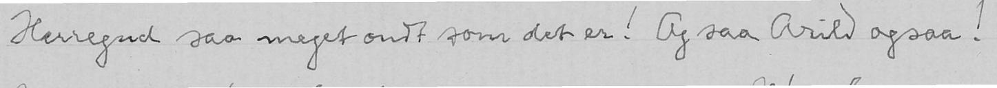Herregud saa meget ondt som det er! Og saa Arild ogsaa!
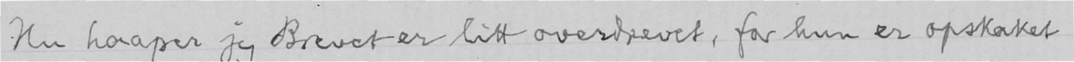Nu haaper jeg Brevet er litt overdrevet, for hun er opskaket
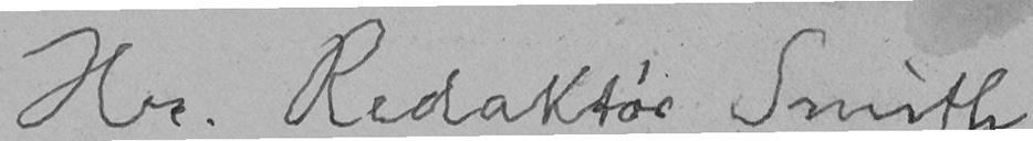Hr. Redaktør Smith
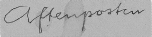Aftenposten
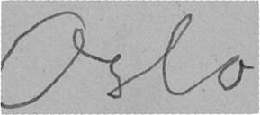Oslo
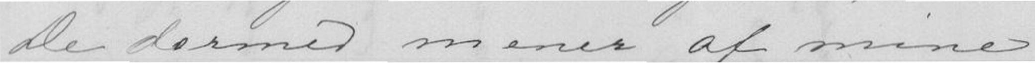De dermed mener af mine
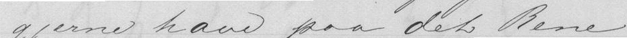gjerne have paa det Rene
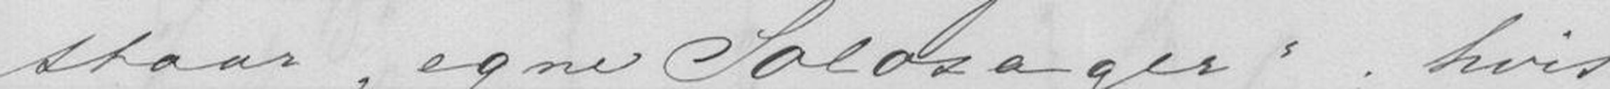staar egne Solosager; hvis
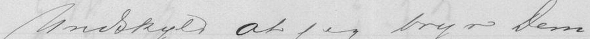Undskyld at jeg bryr Dem
